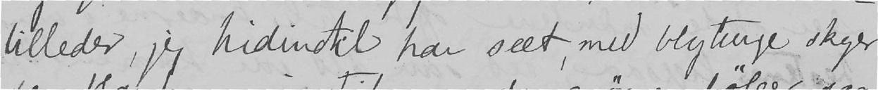billeder, jeg hidindtil har seet, med blytunge skyer
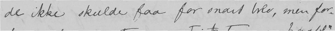de ikke skulde faa for snart brev, men for-
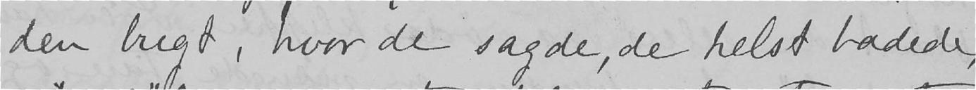den bugt, hvor de sagde, de helst badede,
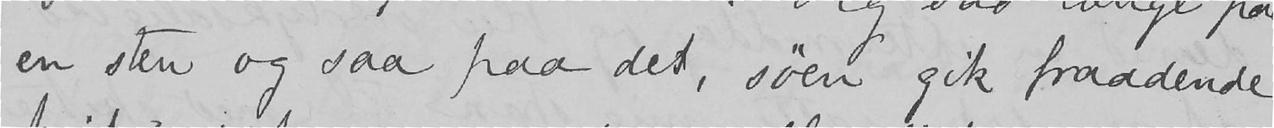en sten og saa paa det, søen gik fraadende
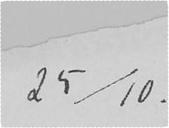25/10.
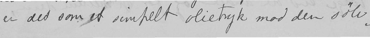er det som et simpelt olietryk mod den sølv-
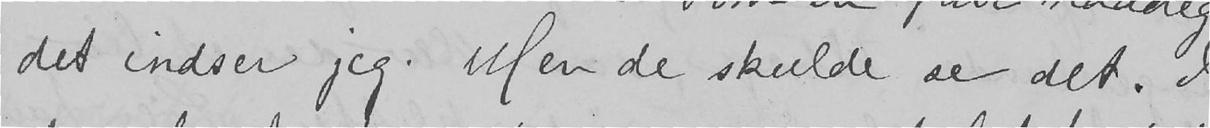det indser jeg. Men de skulde se det. I
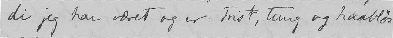di jeg har været og er trist, tung og haabløs.
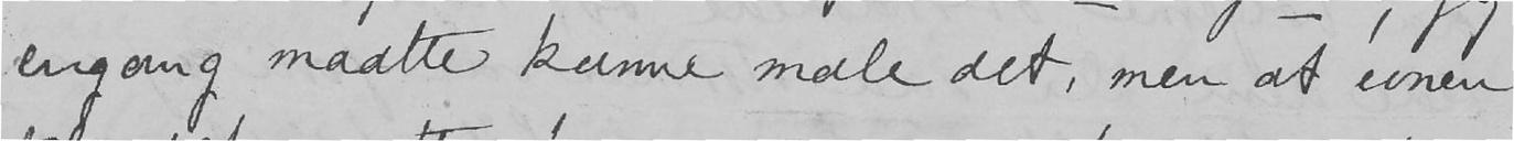engang maatte kunne male det, men at evnen
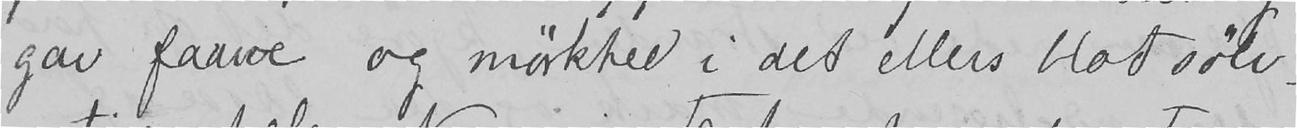gav farve og mørkhed i det ellers blot sølv-
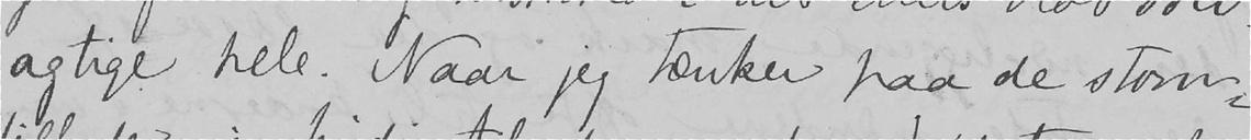agtige hele. Naar jeg tænker paa de storm-
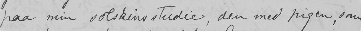paa min solskinsstudie, den med pigen, som
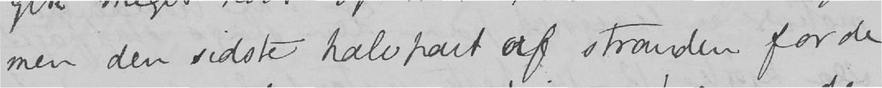men den sidste halvpart af stranden for de
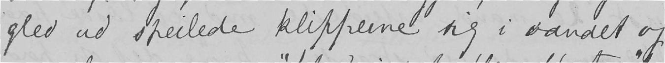gled ud speilede klipperne sig i vandet og
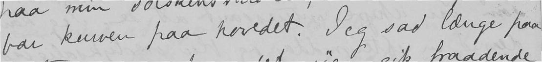bar kurven paa hovedet. Jeg sad længe paa
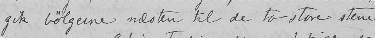gik bølgerne næsten til de to store stene
